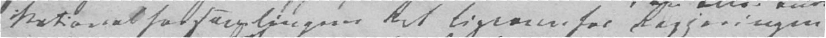Nationalforsamlingens Ret ligeoverfor Regjeringen
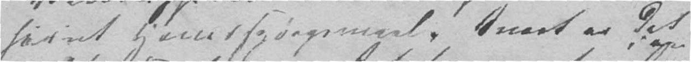hvert Hovedspørgsmaal. Snart er det
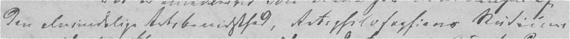den almindelige Retsbevidsthed, Retsphilosophiens Studium
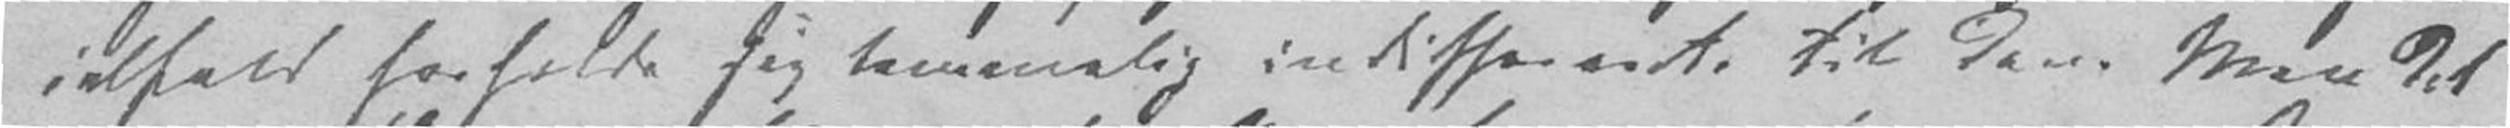ialfald forholde sig temmelig indifferente til den. Men det
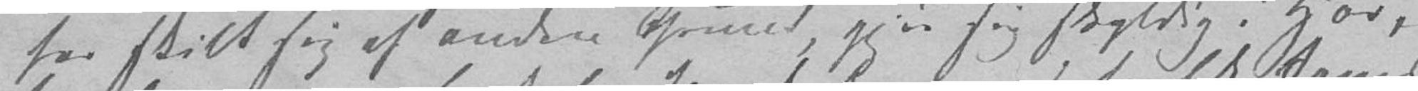har skilt sig af anden Grund, gjør sig skyldig i Hor,
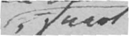, snart
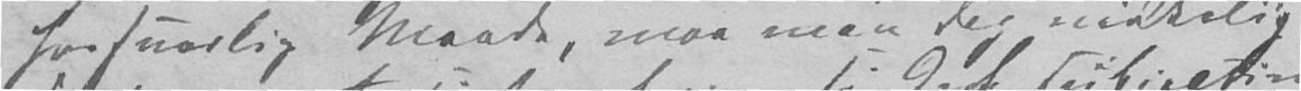forsvarlig Maade, maa man dog virkelig
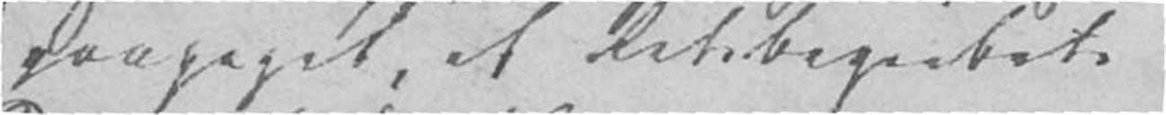paapeget, at Retsbegrebets
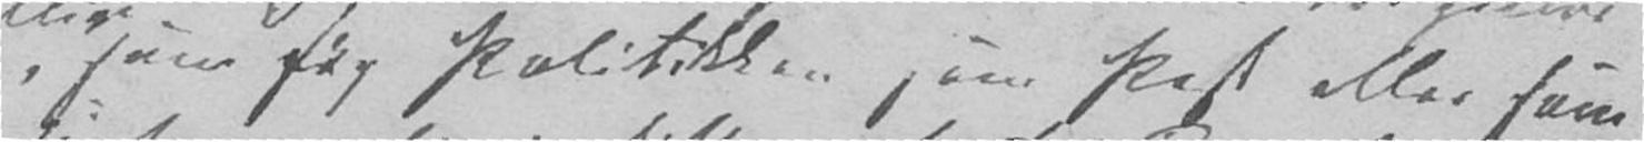, som sky Politikken som Pest eller som
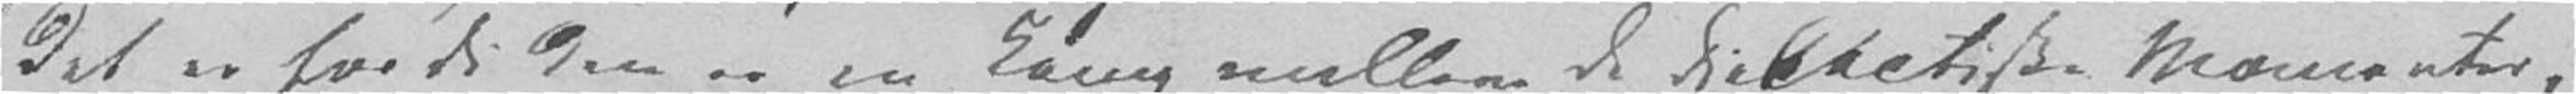Det er fordi den er en Kamp mellem de dialectiske Momenter,
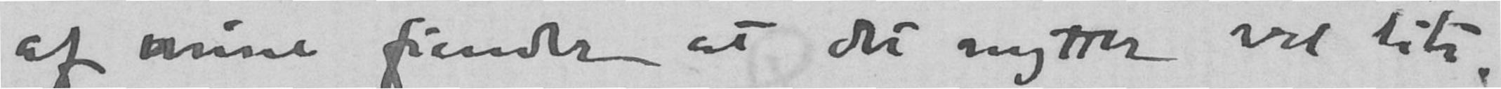af mine fiender at det nytter vel lite.
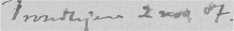Trondhjem 2 nov 07.
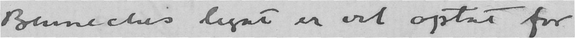Benneches legat er vel optat for
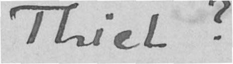Thiel?
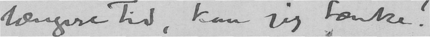længere tid, kan jeg tænke?
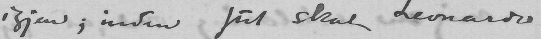igjen; inden jul skal Leonardo
